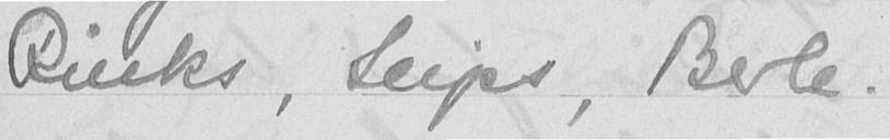Riecks, Seips, Berle.
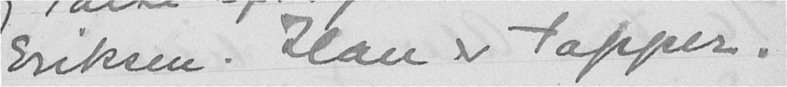Eriksen. Han er tapper.
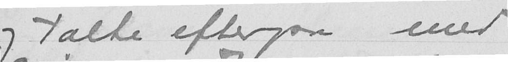og talte efterpaa med
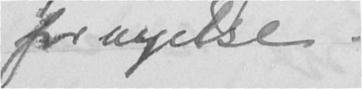fornyelse.
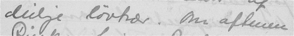deilige løvtrær. Om aftenen
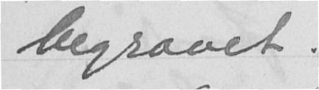begravet.
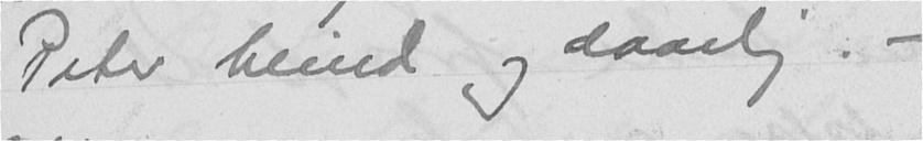Peter blind og daarlig. -
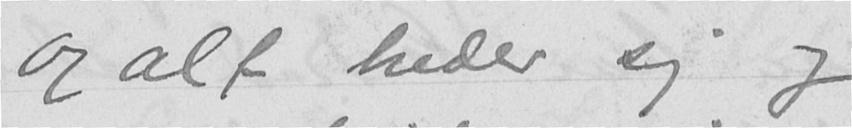Og alt breder sig og
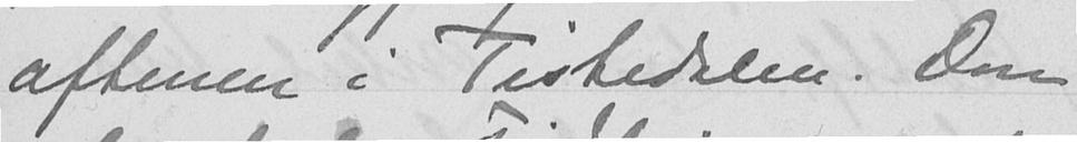aftenen i Tistedalen. Don
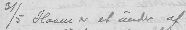31/5 Haven er et under af
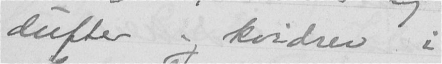dufter og kvidrer i
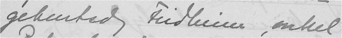geburtsdag Fridheim, onkel
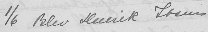1/6 Blev Henrik Ibsen
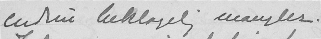endnu beklagelig mangler.
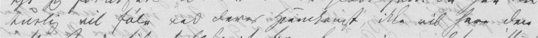turlig vil føle ved deres Hjemkomst, ikke vil have den
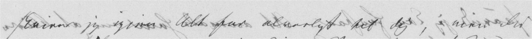skriver jeg igjen for alvorligt til Dig, men Du
